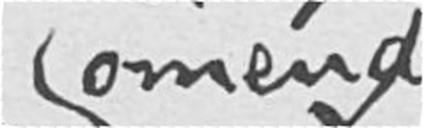omend
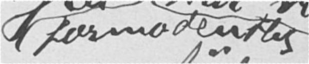formodentlig
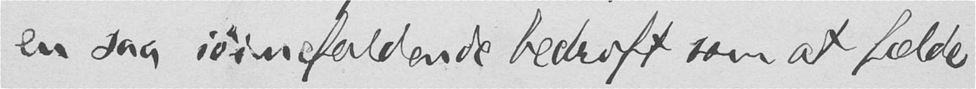en saa iøinefaldende bedrift som at fælde
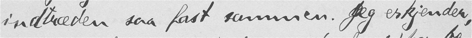indtræden saa fast sammen. Jeg erkjender
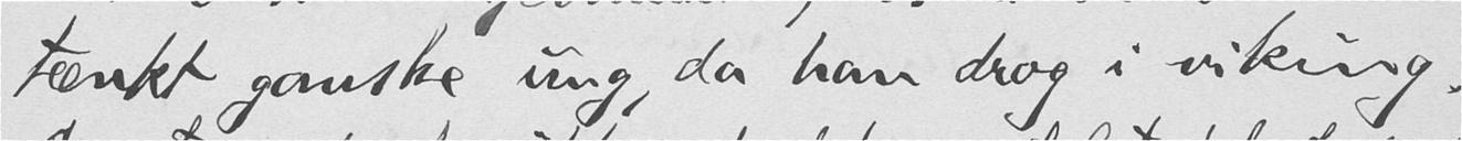tænkt ganske ung, da han drog i viking.
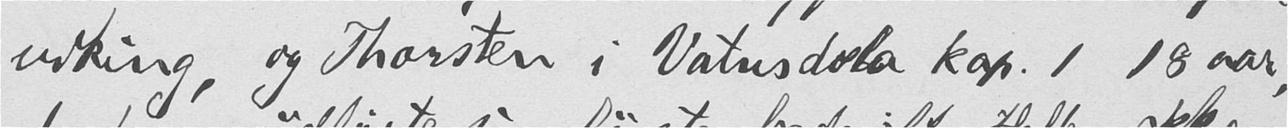viking, og Thorsten i Vatnsdæla kap. 1 18 aar,
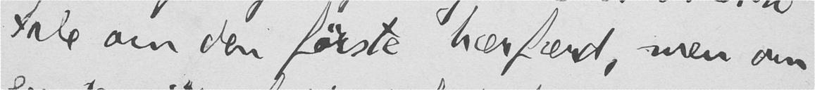tale om den første hærfærd, men om
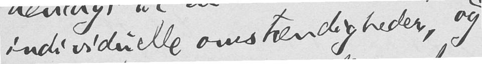individuelle omstændigheder, og
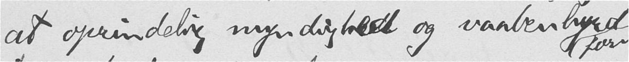at oprindelig myndighed og vaabenbyrd
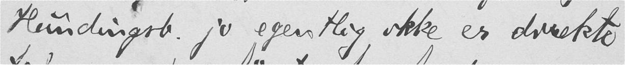Hundingsb. jo egentlig ikke er direkte
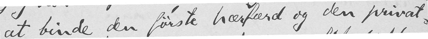at binde den første hærfærd og den privat-
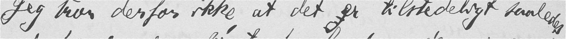Jeg tror derfor ikke, at det er tilstedeligt saaledes
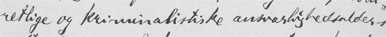retlige og kriminalistiske ansvarlighedsalders
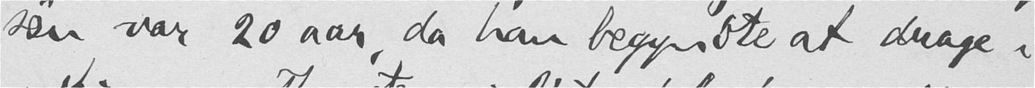søn var 20 aar, da han begyndte at drage i
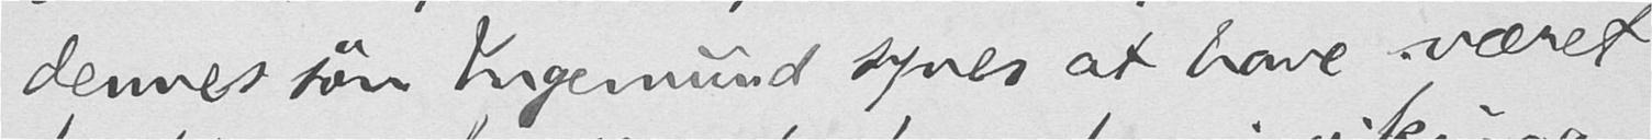dennes søn Ingemund synes at have været
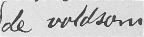de voldsom-
